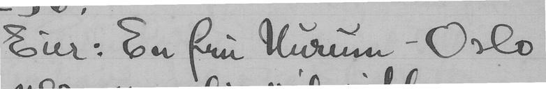Eier: En fu Hurum - Oslo
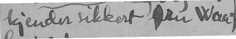kjender sikkert fru Waa-
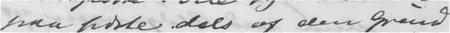paa sidste dels af den grund
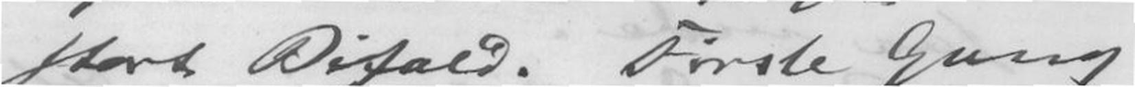stort Bifald. Første Gang
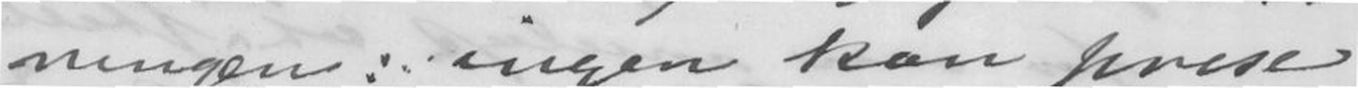ningen: ingen kan prise
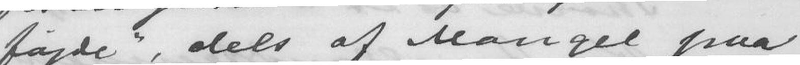føjde", dels af Mangel paa
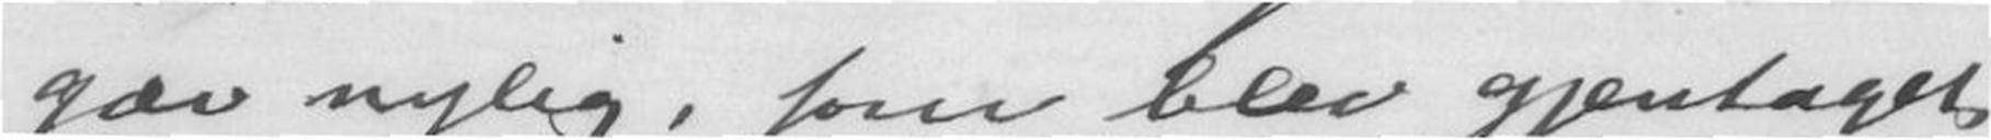gav nylig, somblev gjentaget
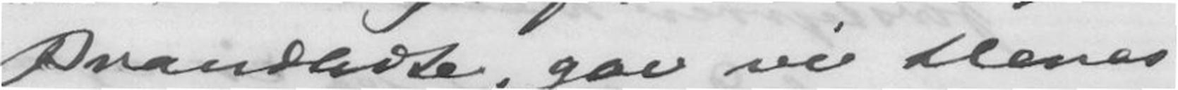Brandledte, gav vi deres
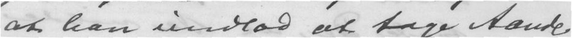at han undlod at tage Aande
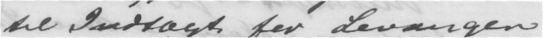til Indtægt for Levanger
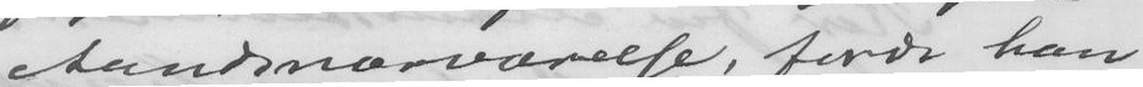Aandsnærværelse, fordi han
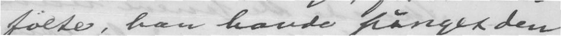følte, han havde sunget den
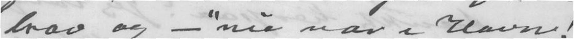brav og - "nu var i Havn!"
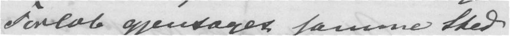Forløb gjentaget samme Sted
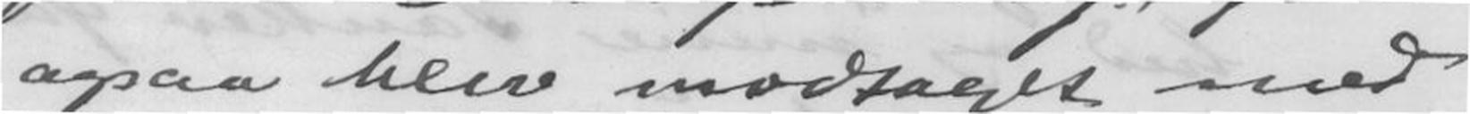ogsaa blev modtaget med
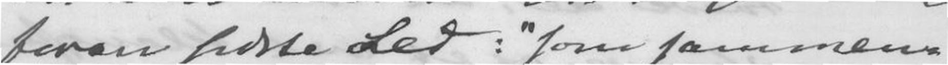foran sidste Led: "som sammen-
